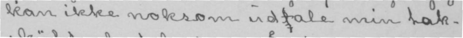kan ikke noksom udtale min tak-
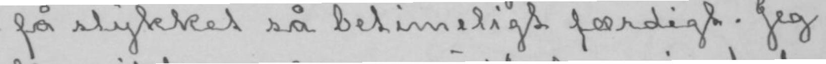få stykket så betimeligt færdigt. Jeg
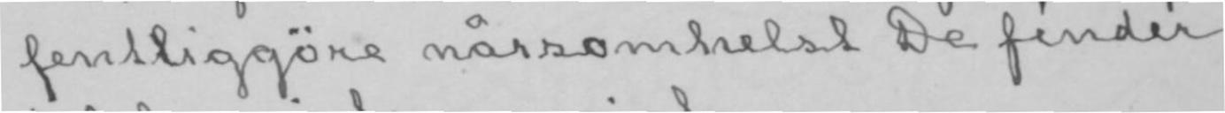fentliggøre nårsomhelst De finder
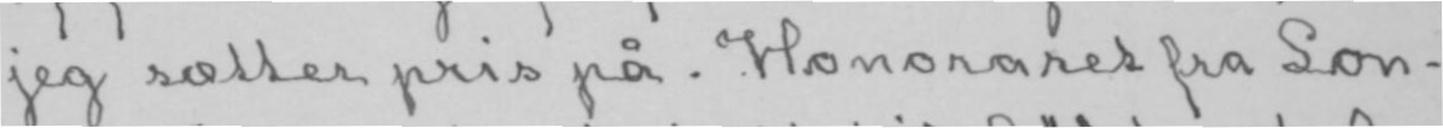jeg sætter pris på. Honoraret fra Lon-
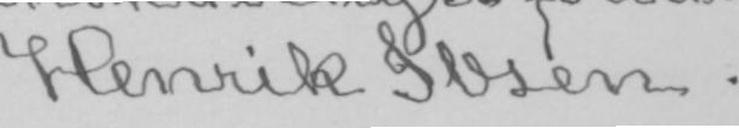Henrik Ibsen.
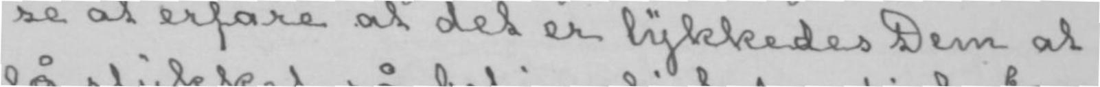se at erfare at det er lykkedes Dem at
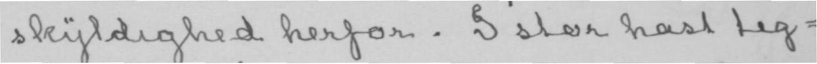skyldighed herfor. I stor hast teg-
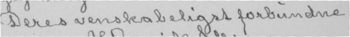Deres venskabeligst forbundne
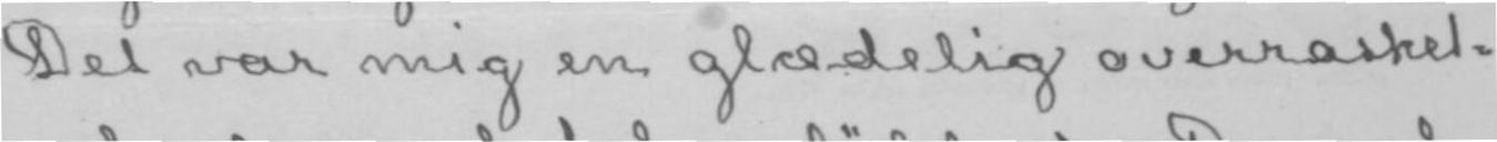Det var mig en glædelig overraskel-
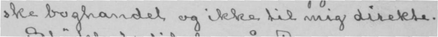ske boghandet og ikke til mig direkte.
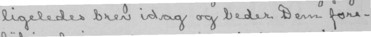ligeledes brev idag og beder Dem fore-
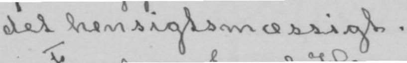det hensigtsmæssigt.
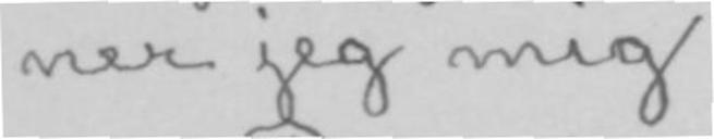ner jeg mig
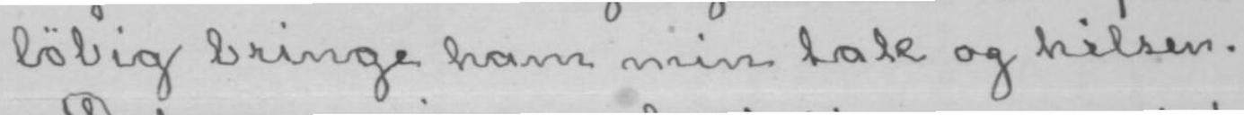løbig bringe ham min tak og hilsen.
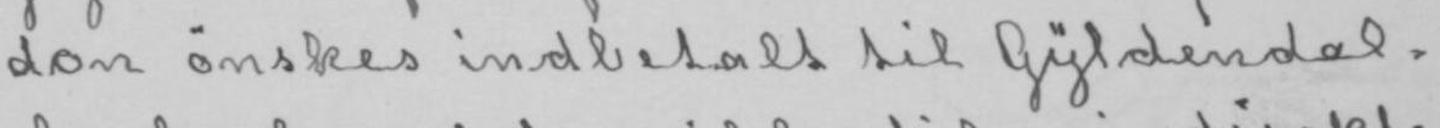don ønskes indbetalt til Gyldendal-
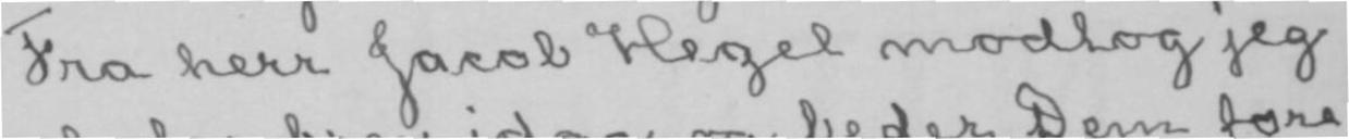Fra herr Jacob Hegel modtog jeg
